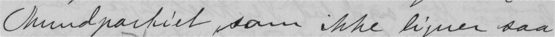Mundpartiet, som ikke ligner saa
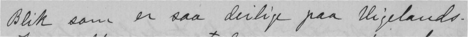Blik som er saa deilige paa Vigelands.
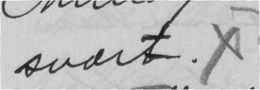svært. x
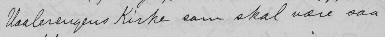Vaalerengens Kirke som skal være saa
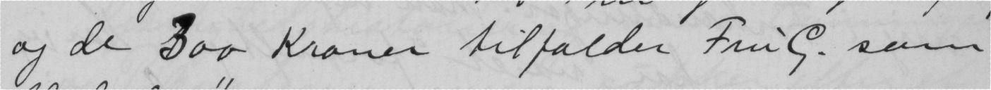og de 300 Kroner tilfalder Fru G. som
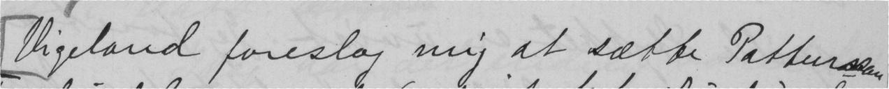Vigeland foreslog mig at sætte Pattursson
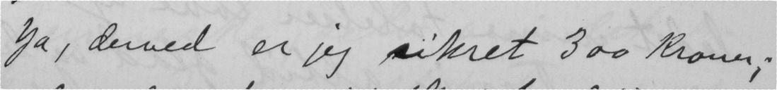Ja, derved er jeg sikret 300 Kroner;
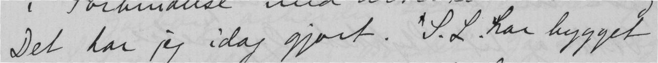Det har jeg idag gjort. S. L. har bygget
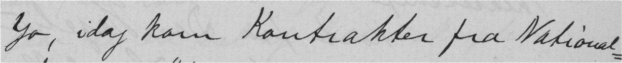Jo, idag kom Kontrakter fra National-
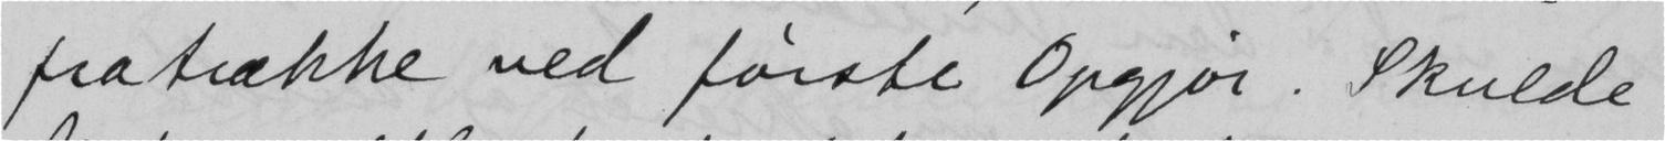fratrække ved første Opgjør. Skulde
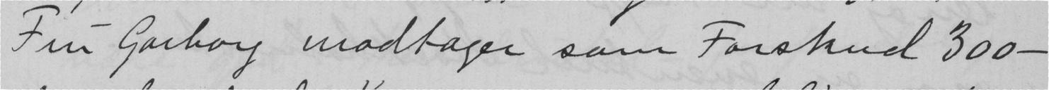Fru Garborg modtager som Forskud 300 -
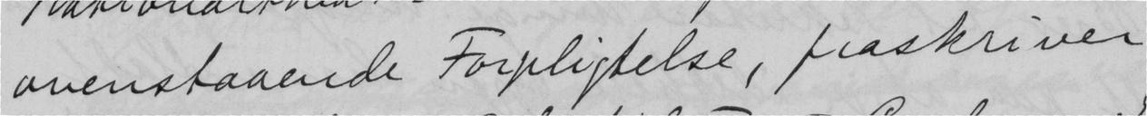ovenstaaende Forpligtelse, fraskriver
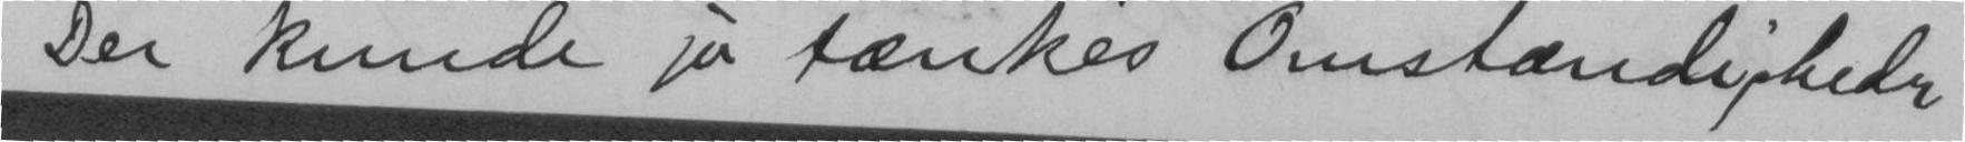Der kunde jo tænkes Omstændigheder
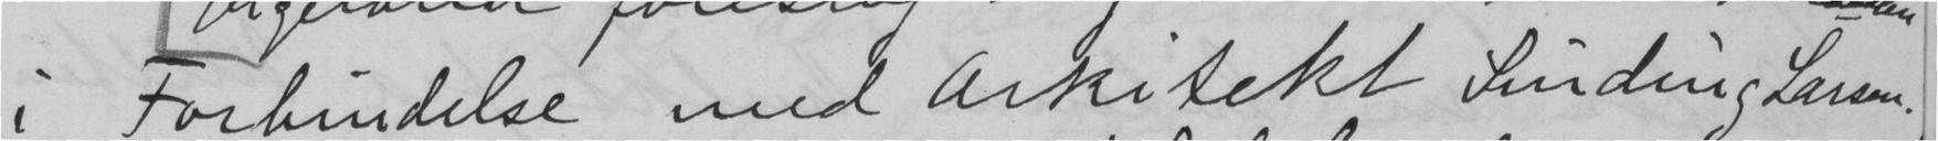i Forbindelse med Arkitekt Sinding Larsen.
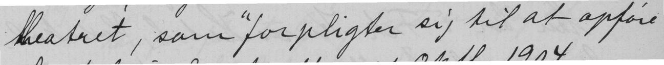theatret, som "forpligter sig til at opføre
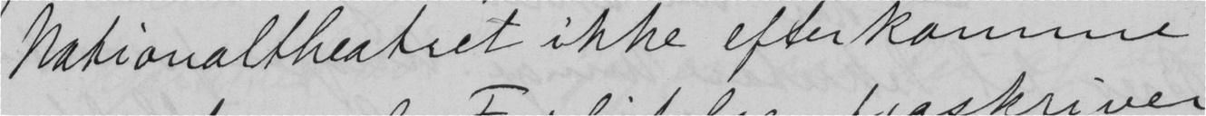Nationaltheatret ikke efterkomme
